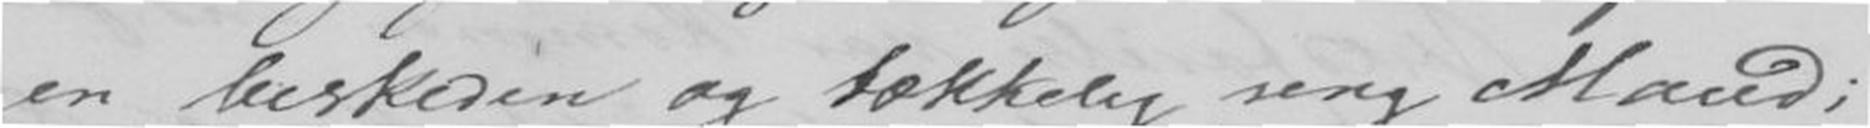en beskeden og tækkelig ung Mand;
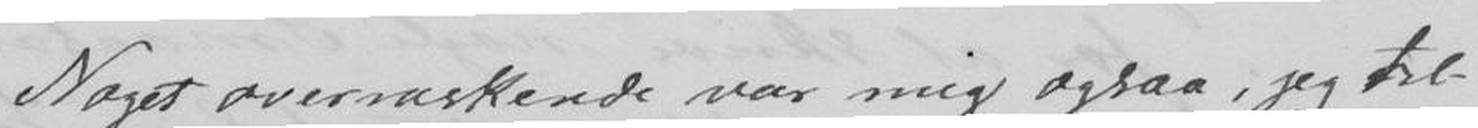Noget overraskende var mig ogsaa, jeg til-
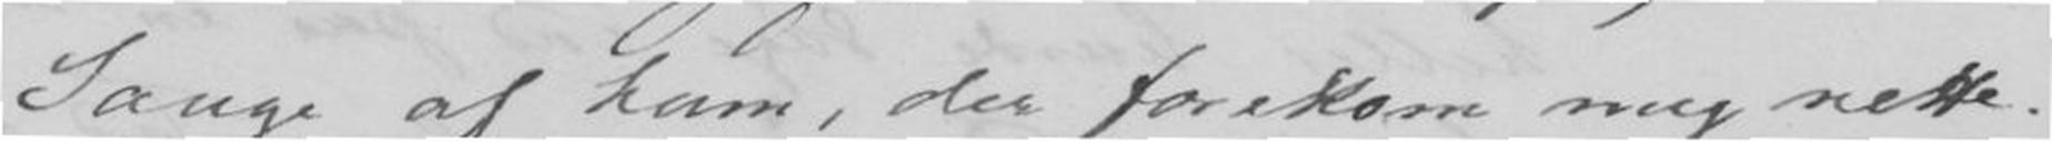Sange af ham, der forekom mig rette.
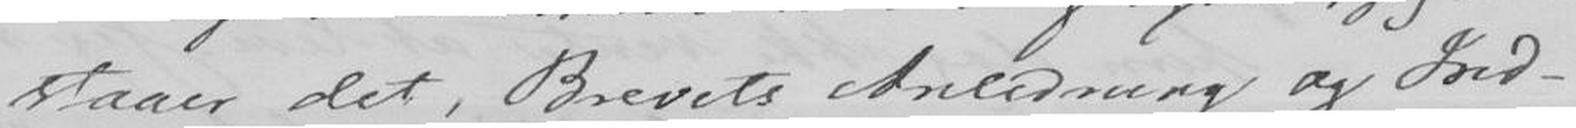staar det, Brevets Anledning og Ind-
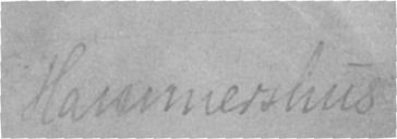Hammerhus
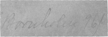(Bornholm 1896)
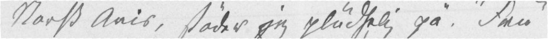Norsk Avis, støder jeg pludselig på «Fru
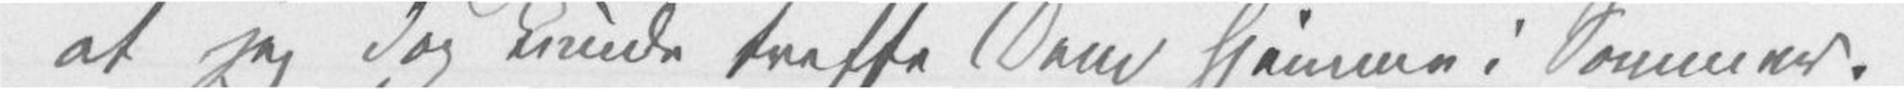at jeg dog kunde treffe Dem Hjemme i Sommer.
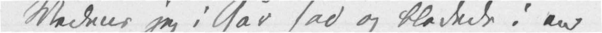Medens jeg i Går sad og bladede i en
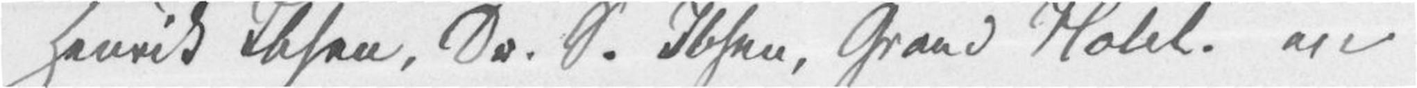Henrik Ibsen, Dr. S. Ibsen, Grand Hotel.» en
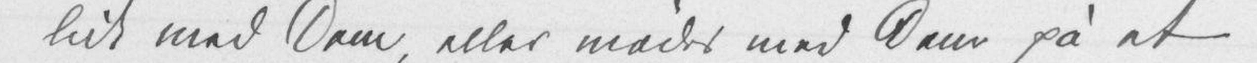lidt med Dem, eller mødes med Dem på et
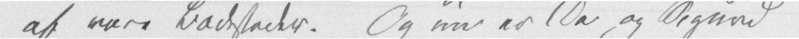af vore Badesteder. Og nu er De og Sigurd
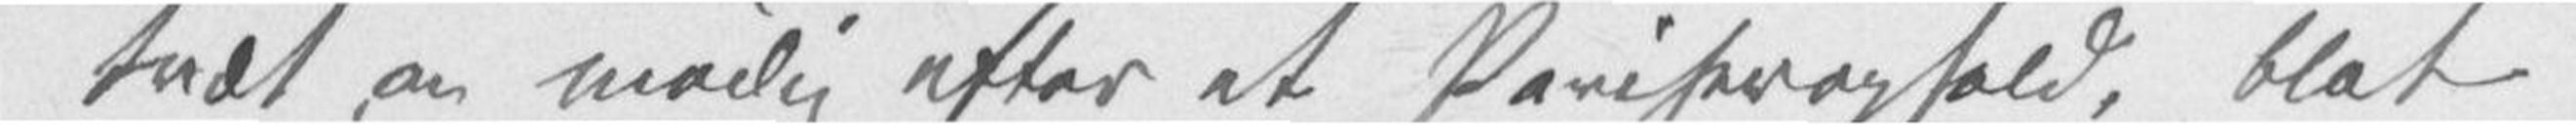træt og mødig efter et Pariserophold, blot
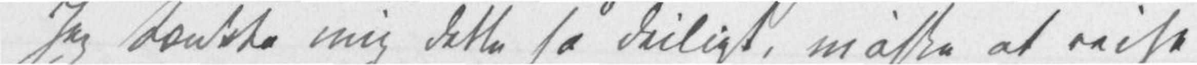Jeg tænkte mig dette så deiligt, måske at reise
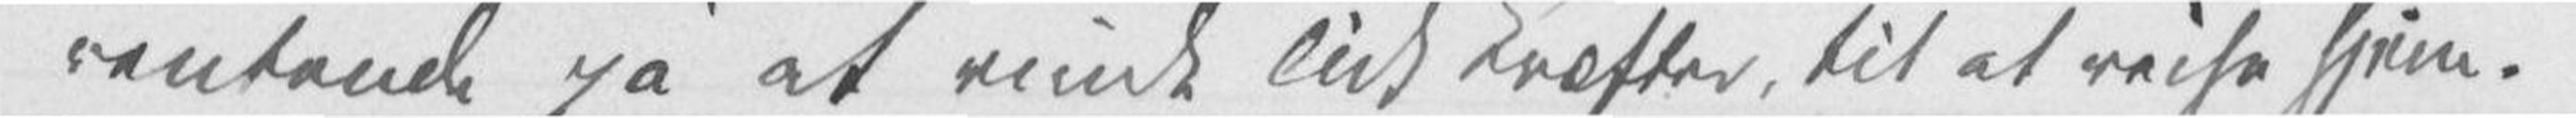ventende på at vinde lidt Kræfter, til at reise Hjem.
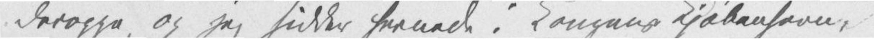deroppe og jeg sidder hernede i Kongens Kjøbenhavn,
